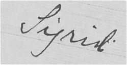Sigrid.
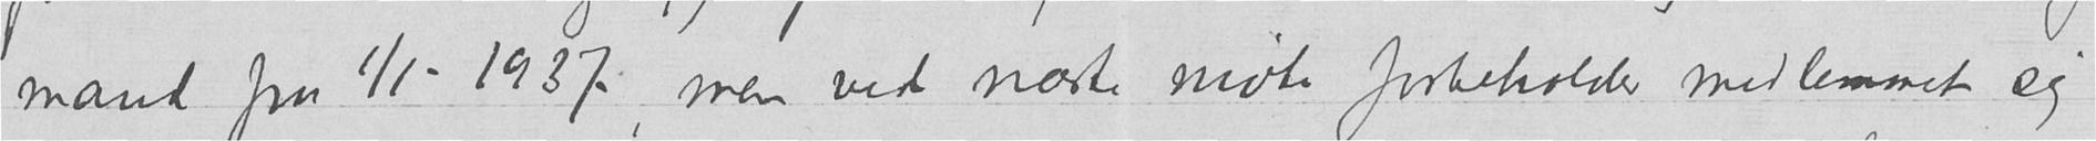mand fra 1/1-1937, men ved næste møte forbeholder medlemmet sig
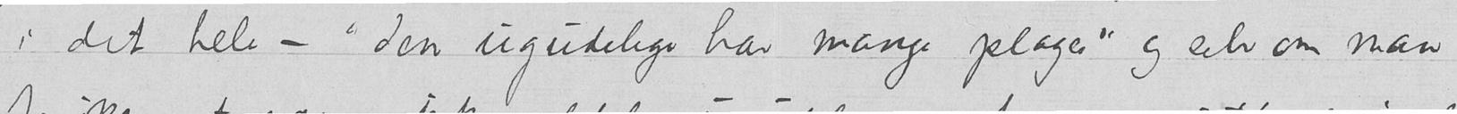i det hele – "den ugudelige har mange plager" og selv om man
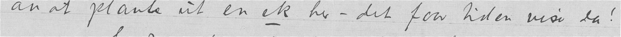an at plante ut en ek her – det faar tiden vise da!
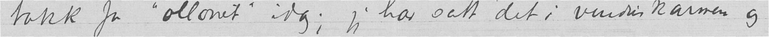takk for "ollonet" idag; jeg har satt det i vinduskarmen og
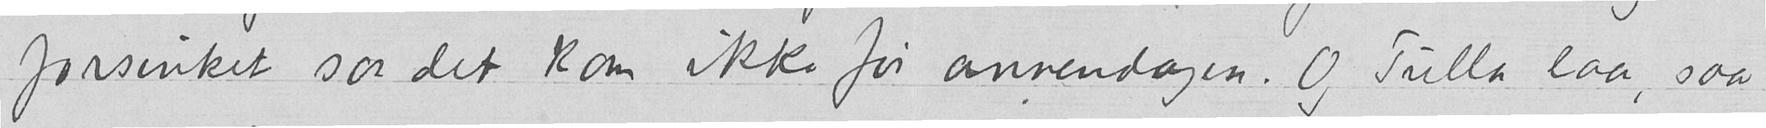forsinket saa det kom ikke før annendagen. Og Tulla laa, saa
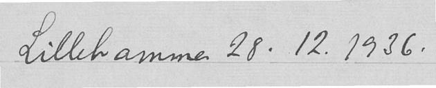Lillehammer 28.12.1936.
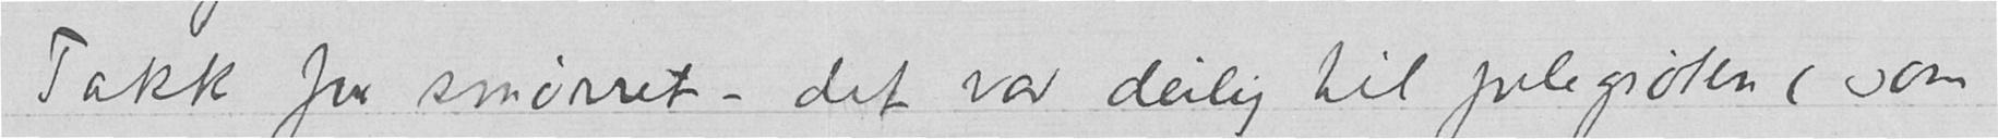Takk for smørret – det var deilig til julegrøten (som
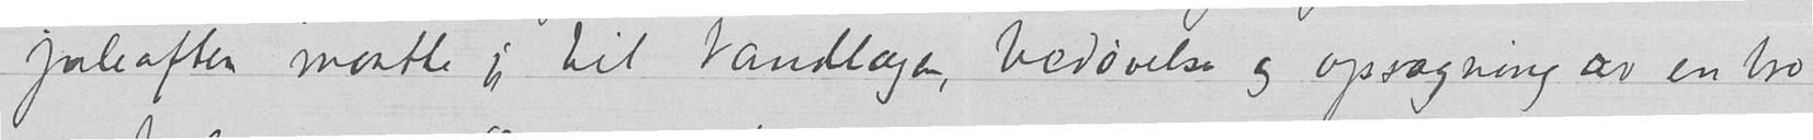juleaften maatte jeg til tandlægen, bedøvelse og opsagning av en bro
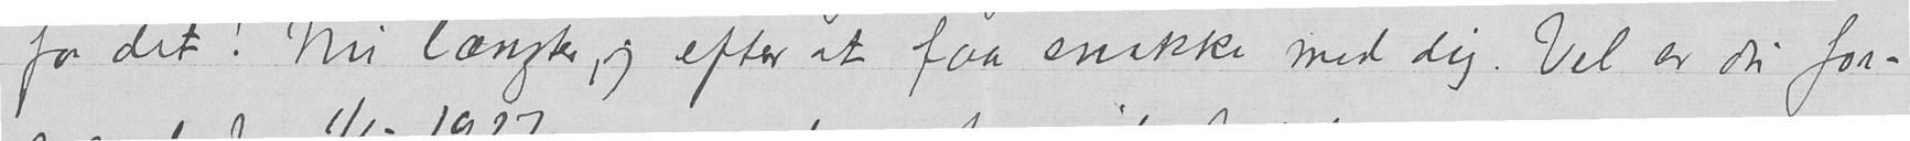for det! Nu længter jeg efter at faa snakke med dig. Vel er du for-
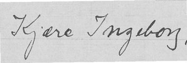Kjære Ingeborg,
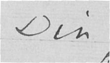Din
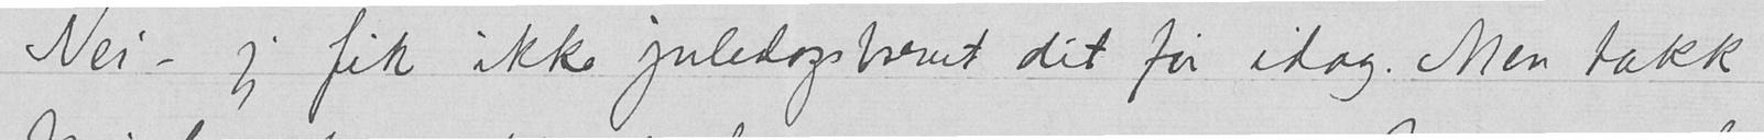Nei – jeg fik ikke juledagsbrevet dit før idag. Men takk
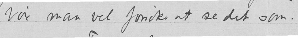bør man vel forsøke at se det som.
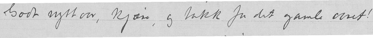Godt nyttaar, kjære, og takk for det gamle aaret!
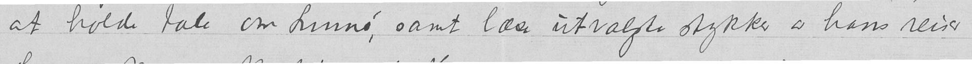at holde tale om Linné, samt læse utvalgte stykker av hans reiser.
